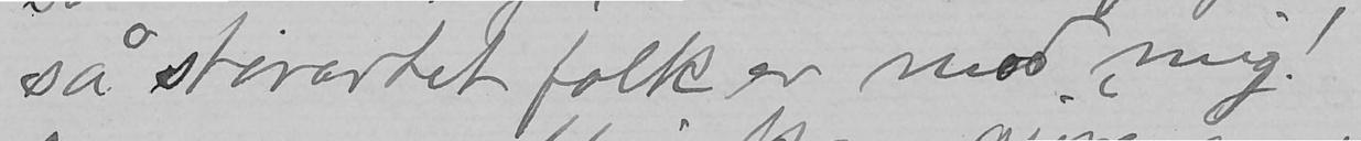så storartet folk er med mig!
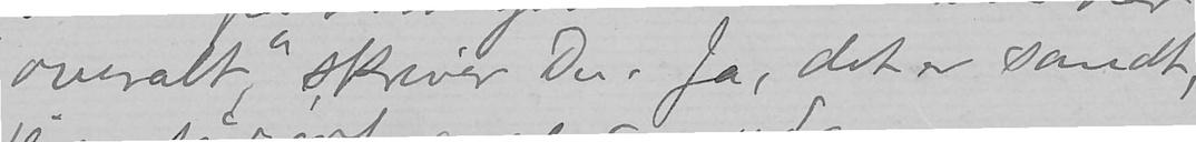overalt," skriver Du. Ja, det er sandt,
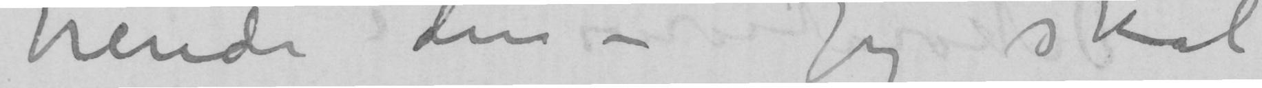hende den – Jeg skal
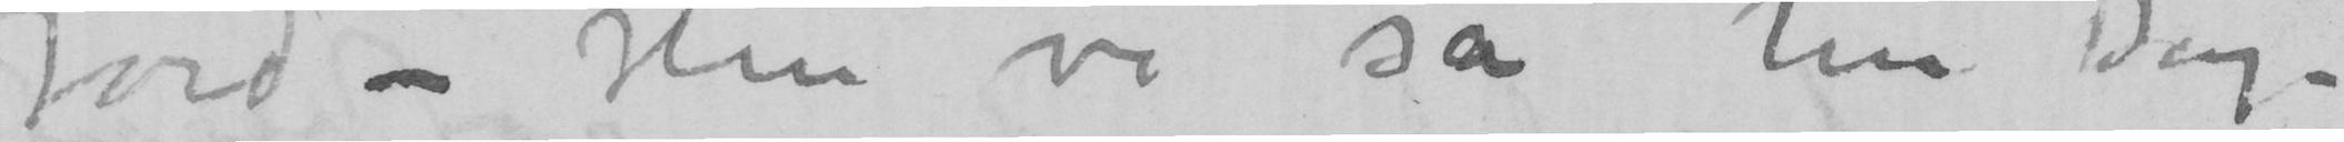Jord – Hun vi så hin Dag
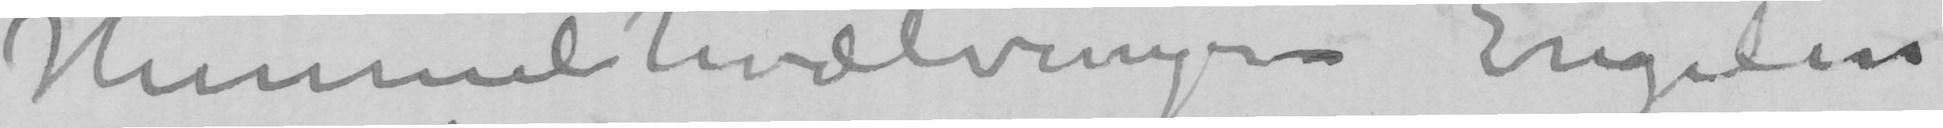Himmelhvælvingen Engelen
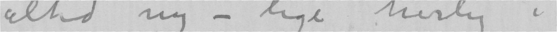altid ny – like herlig i
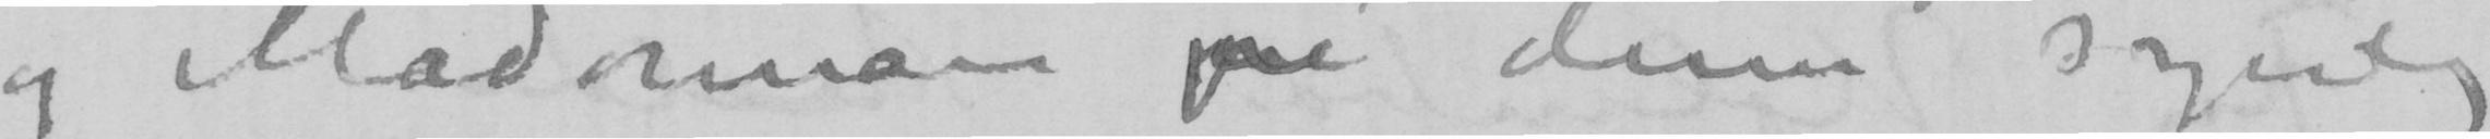og Madonnaen på denne syndige
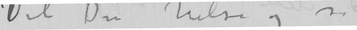Vil Du hilse og si
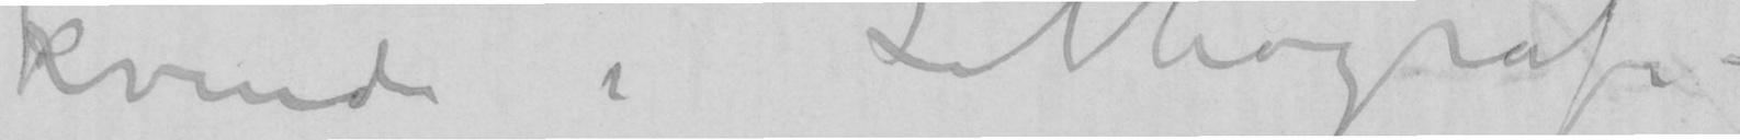kvinde i Litografi –
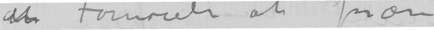aten Fornøielse at forære
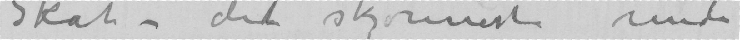Skat – det skjønneste under
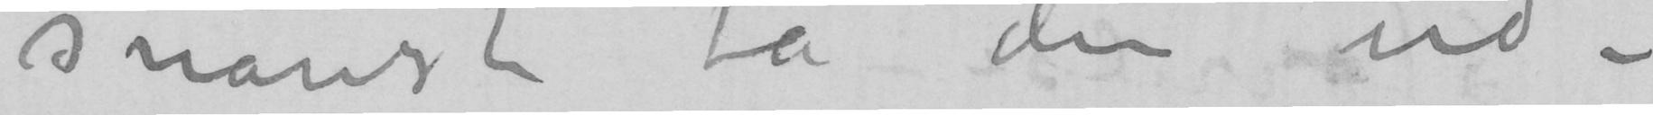snarest ta den ud –
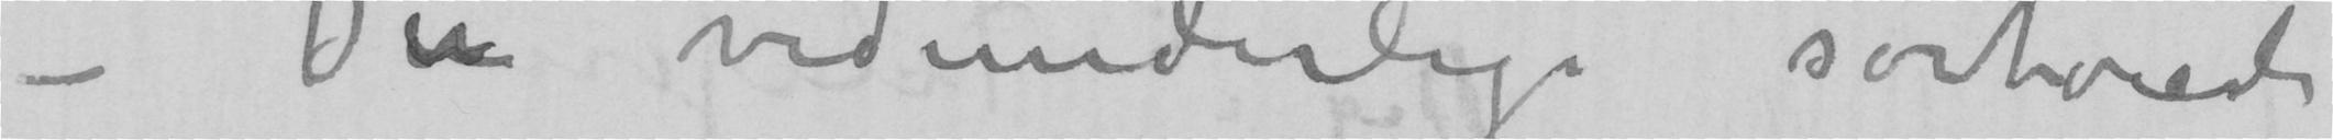– Den vidunderlige sortøiede
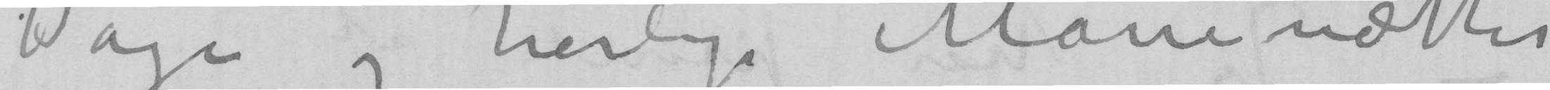Dage og herlige Månenætter
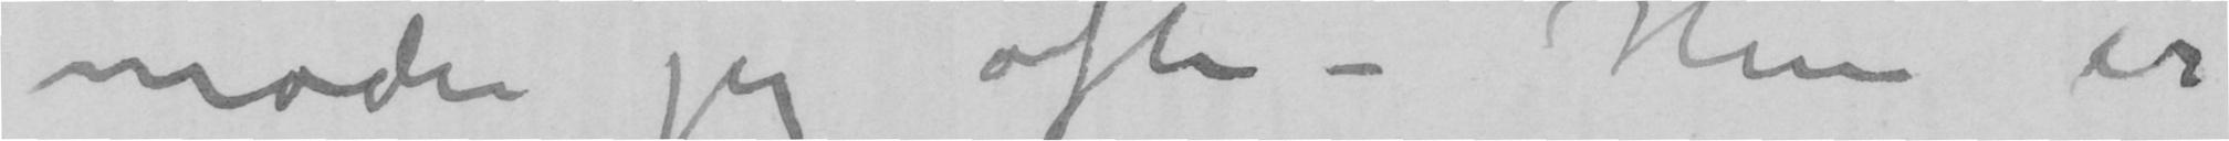møder jeg ofte – Hun er
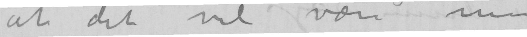at det vil være mig
